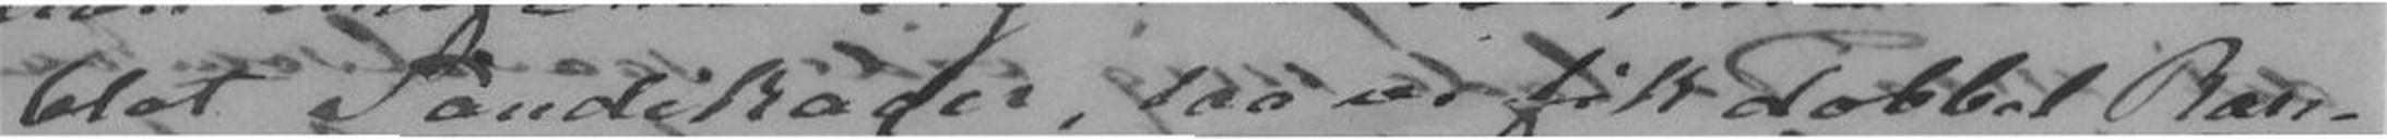blot Pandekager, saa vi fik dobbel Ran-
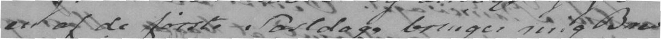en af de første Postdage bringer mig Brev.
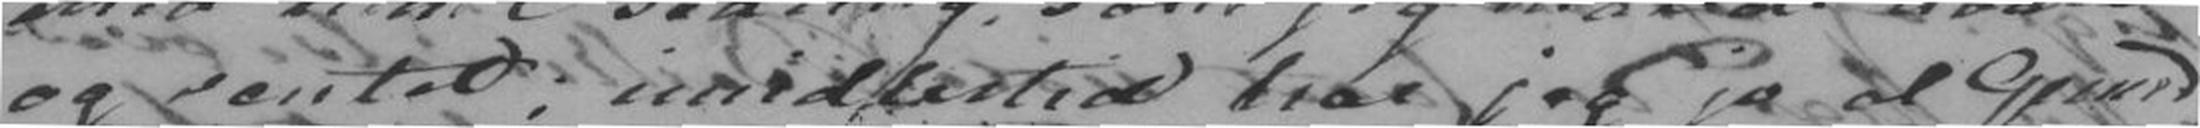og ventet; imidlertid har jeg jo al Grund
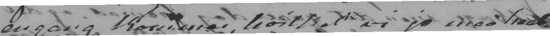engang kommer, hvilket vi jo maa Ind
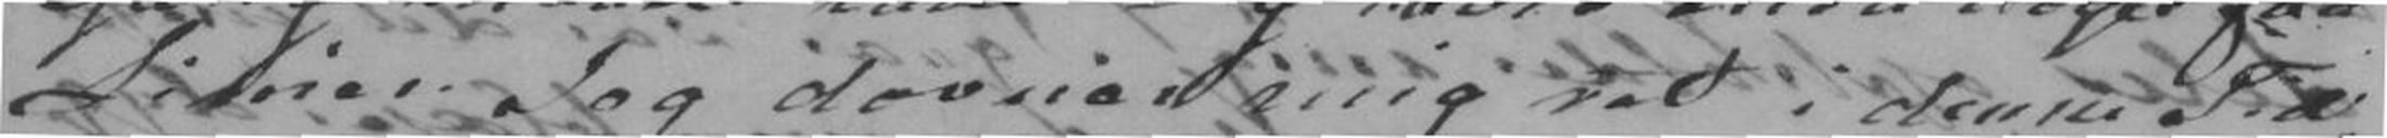Linier. Jeg dovner mig ret i denne Tid,
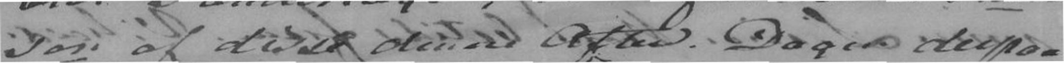son af disse denne Aften. Dagen derpaa
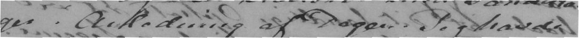ger i Anledning af Dagen. Jeg havde
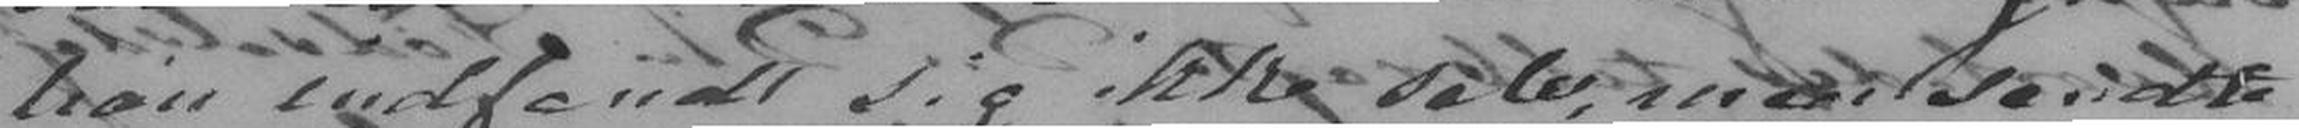han indfandt sig ikke selv, men sendte
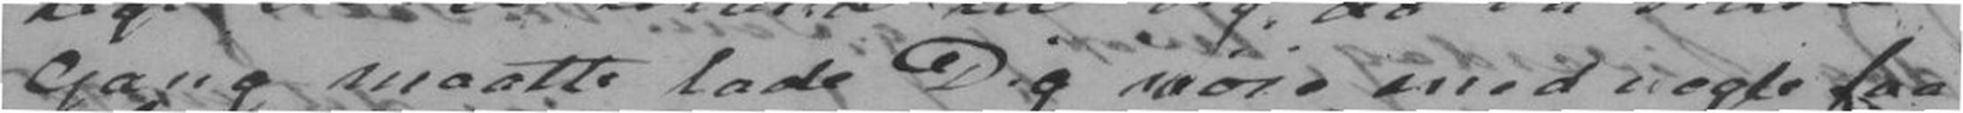gang maatte lade Dig nøie med nogle faa
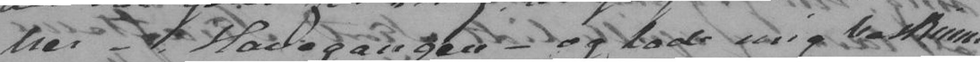her i Havegangen - og lade mig beskinne
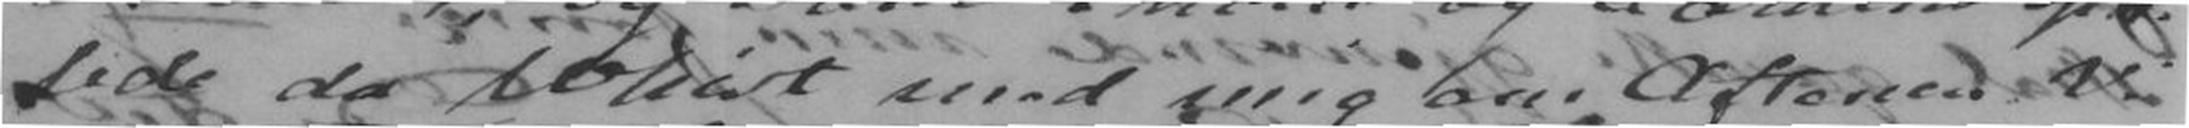lede da Whist med mig om Aftene. Vi
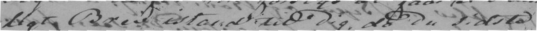ligt Brev istand til Dig, da Du sidste
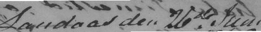Landaas den 26de Juni
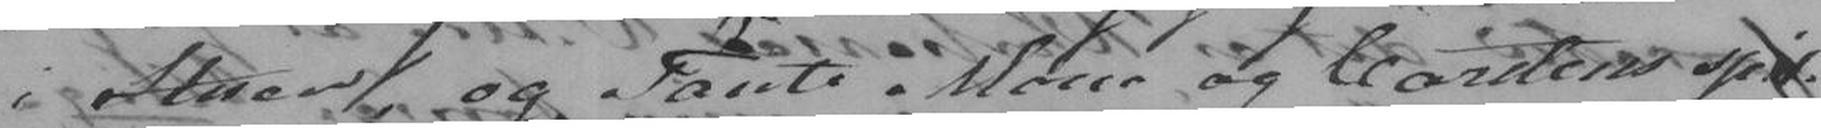i Stuen, og Tante Mone og Carsten spil-
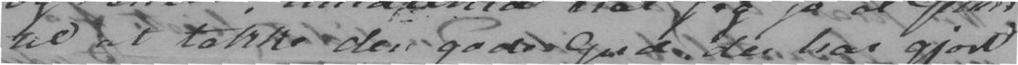til at takke den gode Gud der har gjort
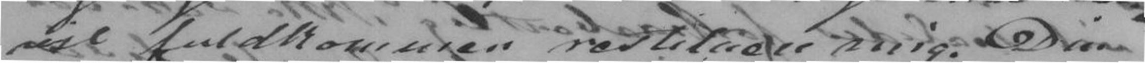vil fuldkommen reststiturere mig. Din
